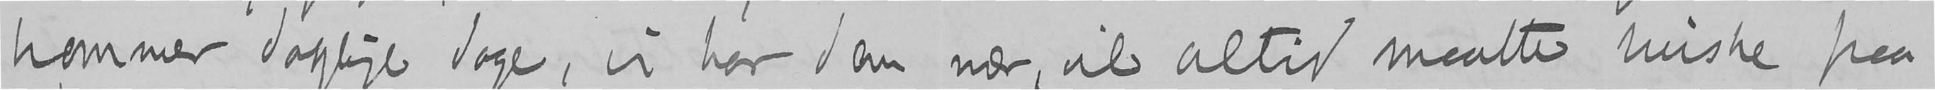kommer daglige dage, vi har dem nær, vil altid maatte huske paa
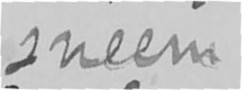sneen
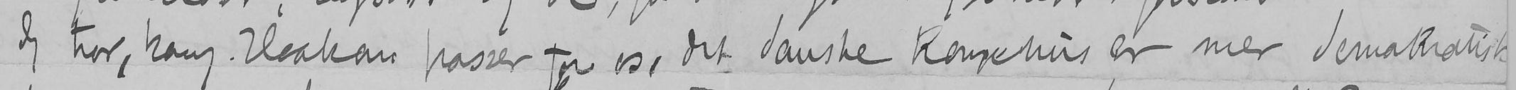Jeg tror, kong Haakon passer for os, det danske kongehus er mer demokratisk
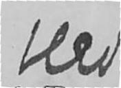blev
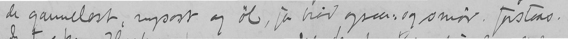de gammelost, mysost og øl, ja brød ogsaa og smør forstaas.
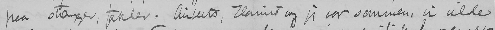paa stænger, fakler. Auberts, Harriet og jeg var sammen, vi vilde
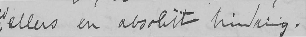ellers en absolut hindring.
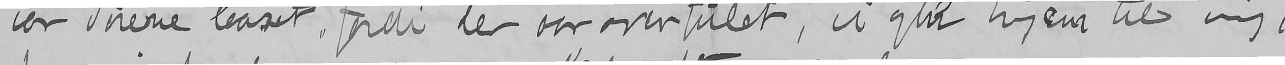var dørene laaset, fordi der var overfuldt, vi gik hjem til mig,
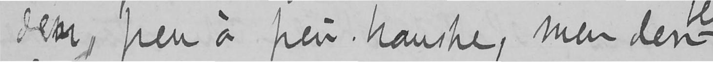dem, peu à peu kanske, men den
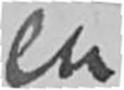en
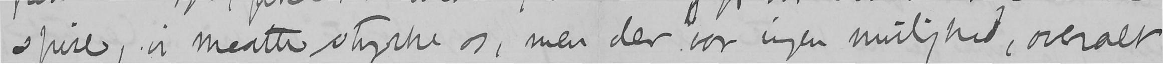spise, vi maatte styrke os, men der var ingen mulighed, overalt
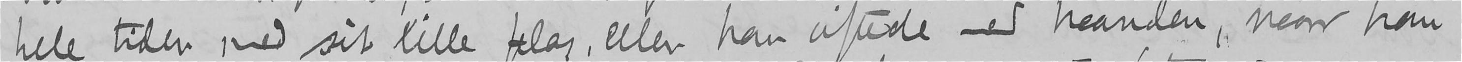hele tiden med sit lille flag, eller han viftede med haanden, naar han
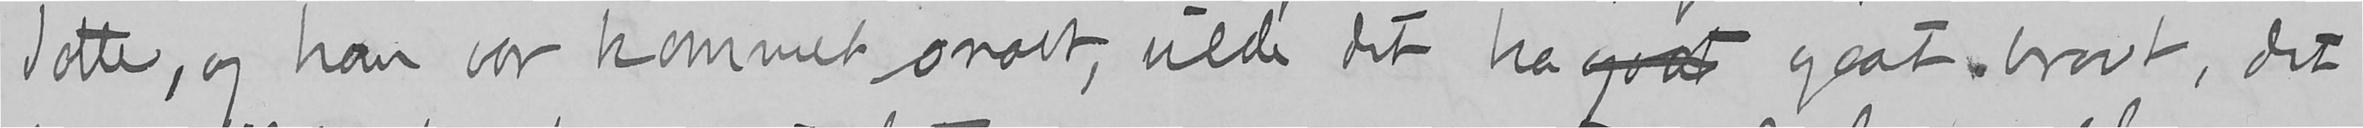dotte, og han var kommet snart, vilde det ha godt gaat bravt, det
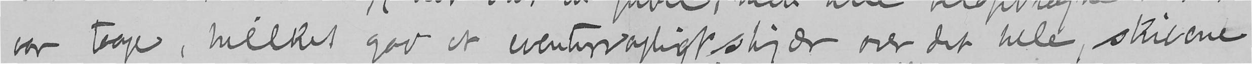var taage, hvilket gav et eventyragtigt skjær over det hele, skibene
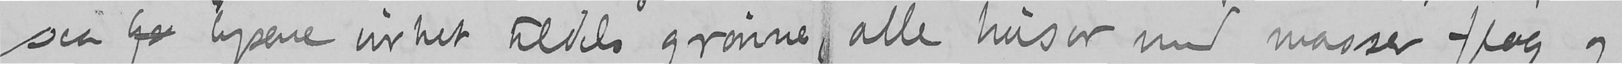saa pa lysene virket tildels grønne, alle huser med masser flag og
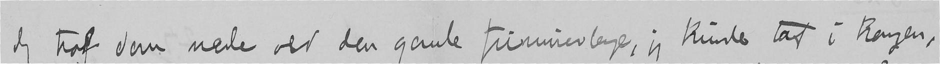Jeg traf dem nede ved den gamle frimurerloge, jeg kunde tat i kongen,
